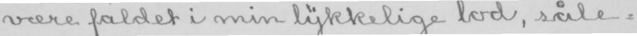være faldet i min lykkelige lod, såle-
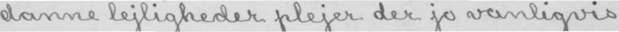danne lejligheder plejer der jo vanligvis
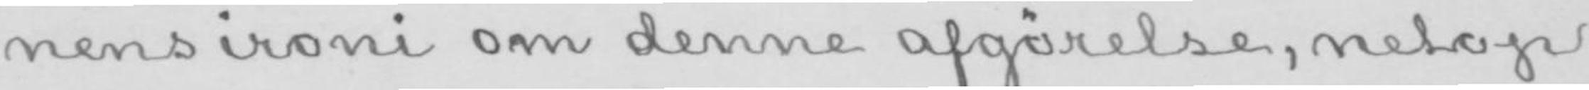nens ironi om denne afgørelse, netop
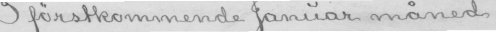I førstkommende Januar måned
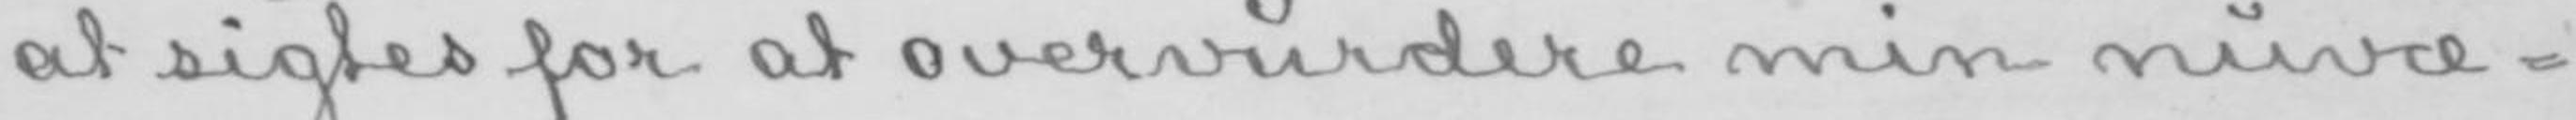at sigtes for at overvurdere min nuvæ-
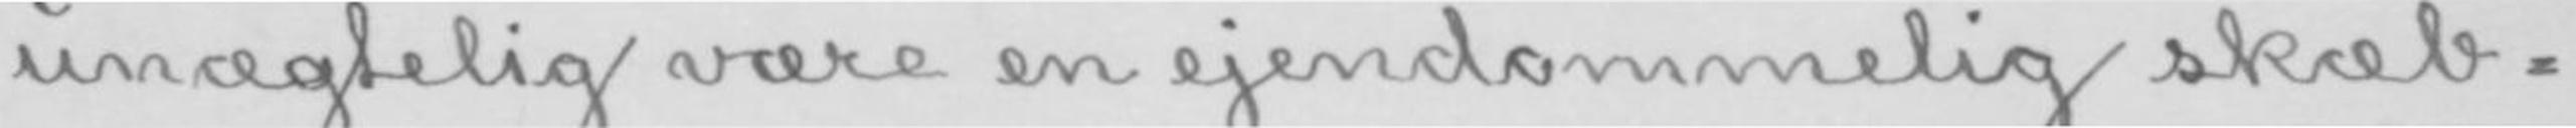unægtelig være en ejendommelig skæb-
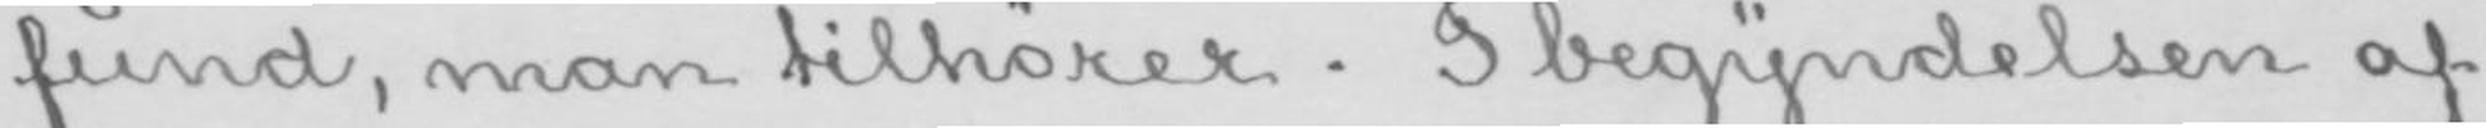fund, man tilhører. I begyndelsen af
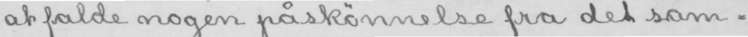at falde nogen påskønnelse fra det sam-
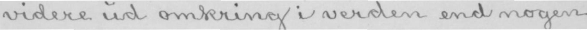videre ud omkring i verden end nogen
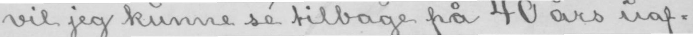vil jeg kunne sé tilbage på 40 års uaf-
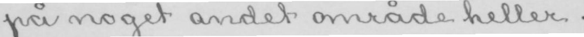på noget andet område heller.
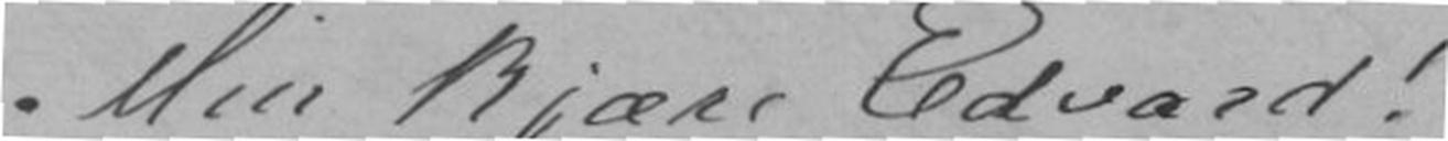Min kjære Edvard!
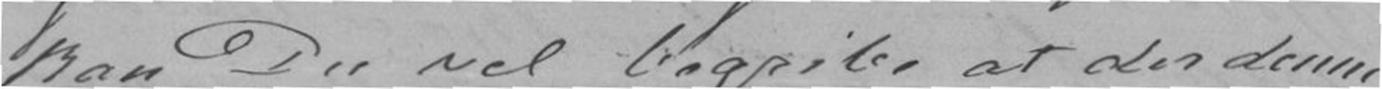kan Du vel begribe at der denne
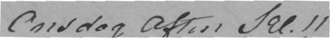Onsdag Aften Kl.11
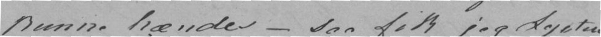kunne hænde - saa fik jeg Lysten
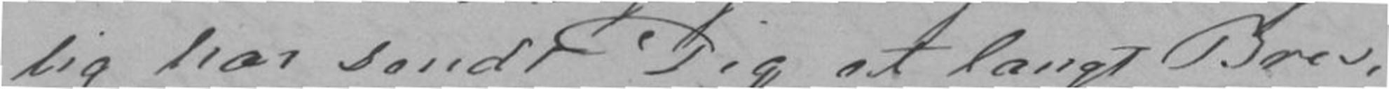lig har sendt Dig et langt Brev,
